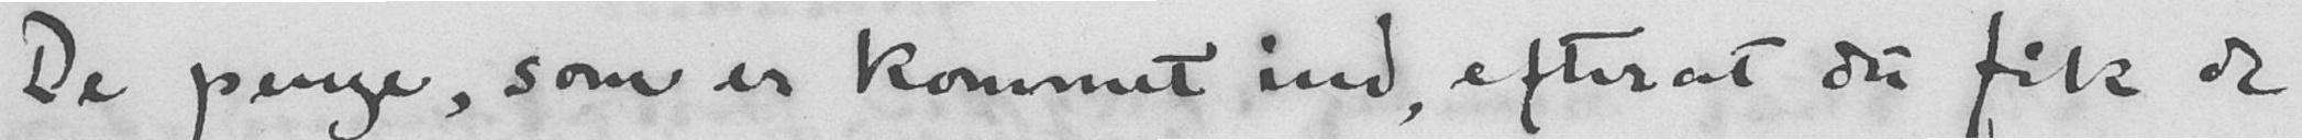De penge, som er kommet ind, efterat du fik de
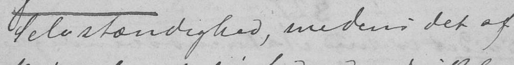Selvstændighed, medens det af
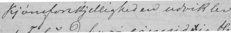Kjønsforskjelligheden udvikler
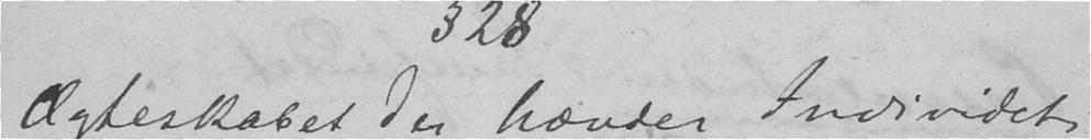Ægteskabet der hævder Individets
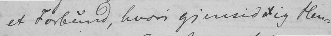et Forbund, hvori gjensidig Hen-
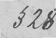§ 28
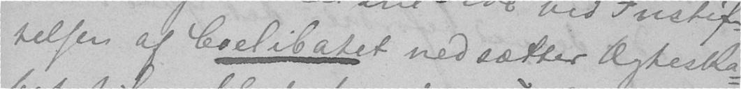telsen af Coelibatet nedsætter Ægteska-
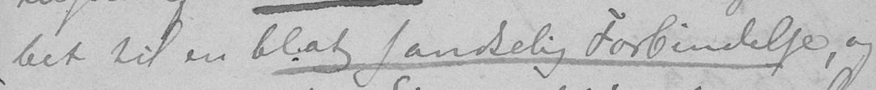bet til en blot sandselig Forbindelse, og
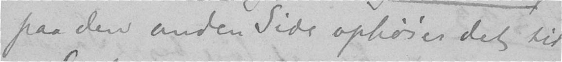paa den anden Side ophøier det til
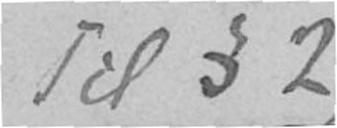Til § 29
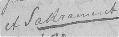et Sakramente
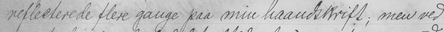reflecterede flere gange paa min haandskrift; men ved
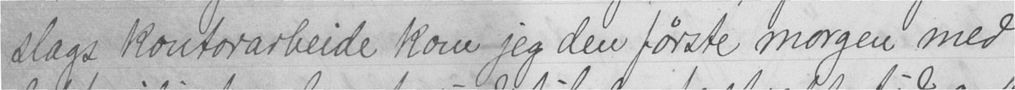slags kontorarbeide kom jeg den første morgen med
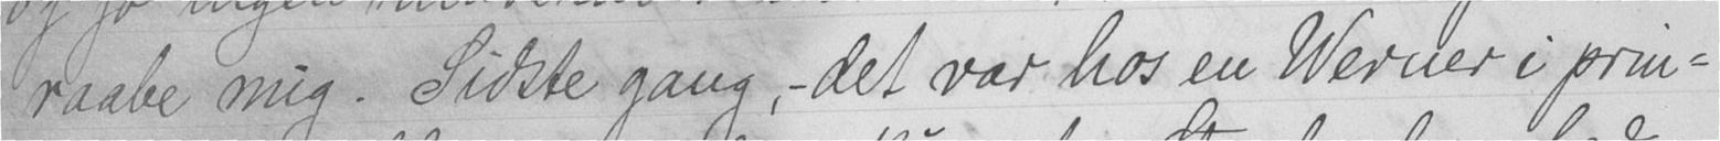raabe mig. Sidste gang, - det var hos en Werner i prin-
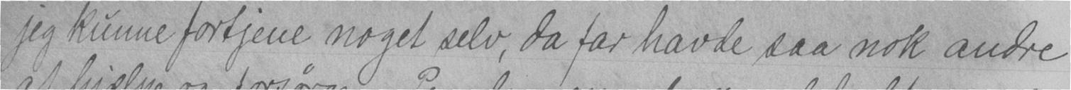jeg kunne fortjene noget selv, da far havde saa nok andre
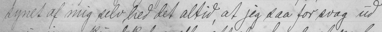synet af mig selv hed det altid, at jeg saa for svag ud
